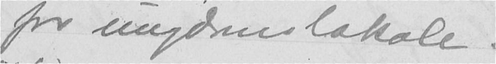for ungdomslokale.
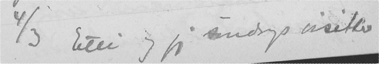4/3 Etti og jeg søndagsvisitter
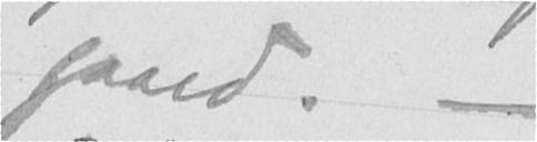gaard. -
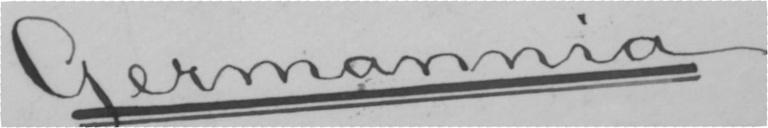Germannia.
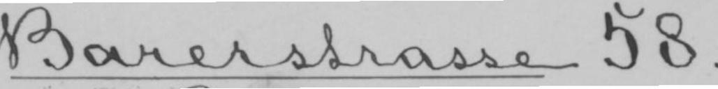Barerstrasse 58.
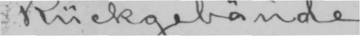Rückgebäude.
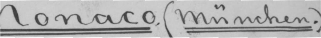Monaco. (München.)
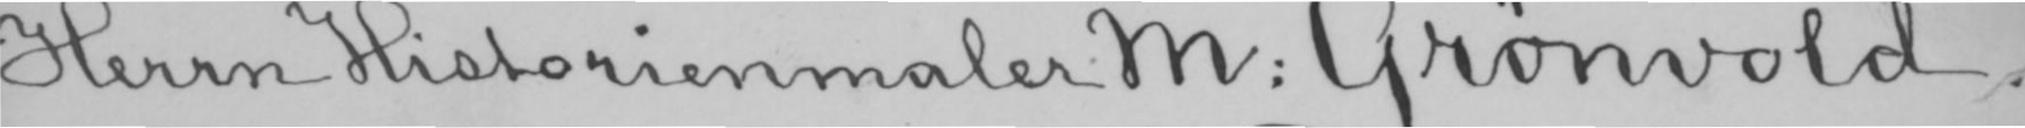Herrn Historienmaler M: Grønvold.
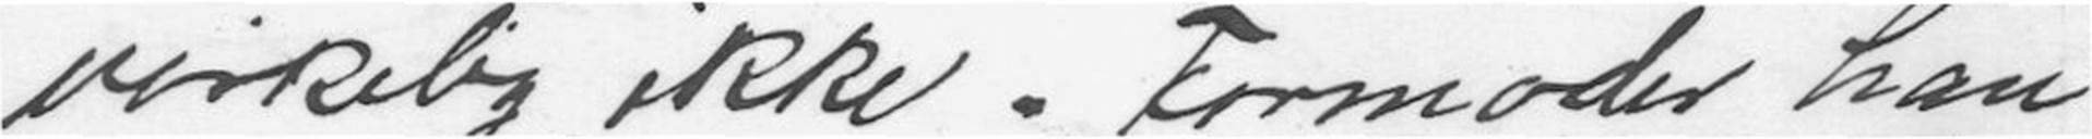virkelig ikke. Formoder han
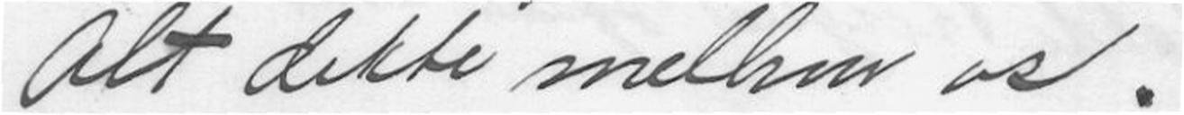Alt dette mellem os.
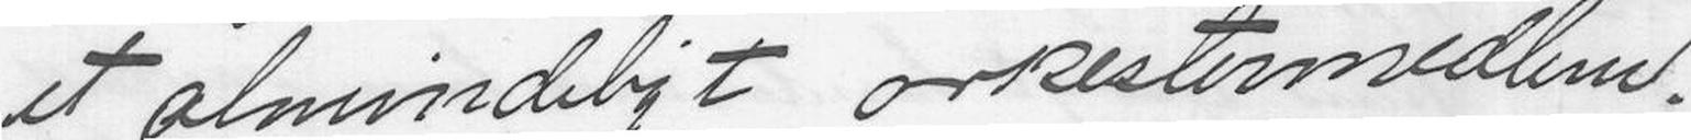et almindeligt orkestermedlem
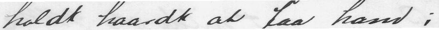holdt haardt at faa ham;
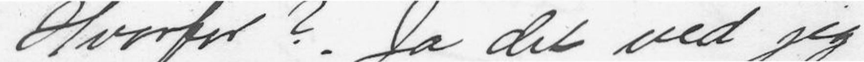Hvorfor? Ja det ved jeg
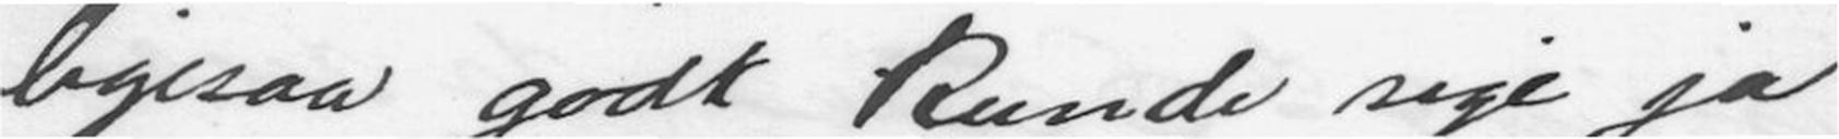ligesaa godt kunde sige ja
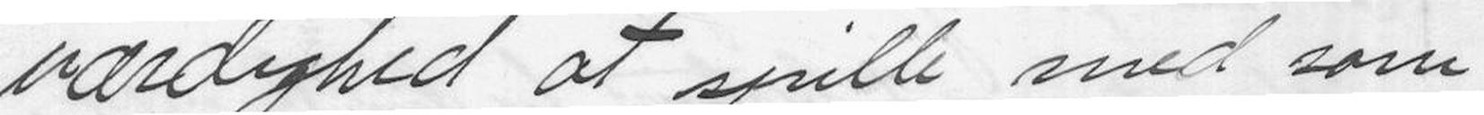værdighed at spille med som
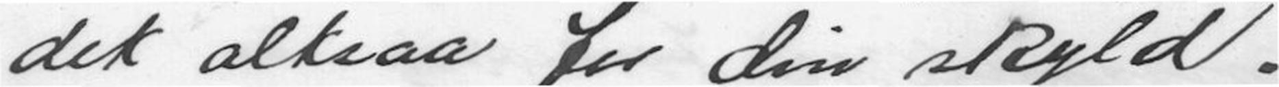det altsaa for din skyld.
